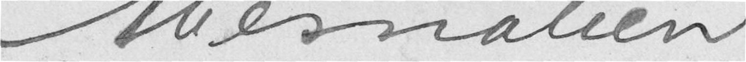Mesnalien
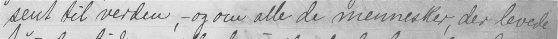sent til verden, - og om alle de mennesker, der levede
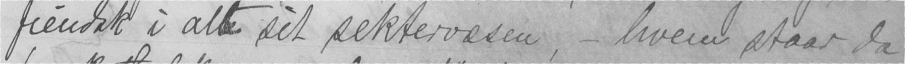fiendsk i alt sit sektervæsen, - hvem staar da
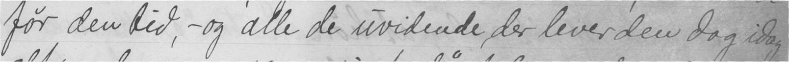før den tid, - og alle de uvidende, der lever den dag idag
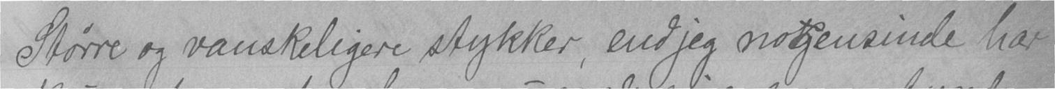Større og vanskeligere stykker, end jeg nogensinde har
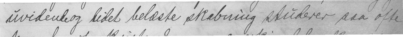uvidende og lidet belæste skabning studerer saa ofte
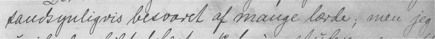sandsynligvis besvaret af mange lærde; men jeg
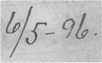6/5-96.
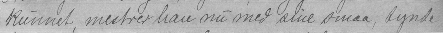kunnet, mestrer han nu med sine smaa, tynde
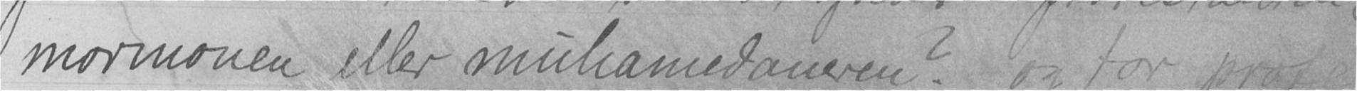mormonen eller muhamedaneren? og for prote-
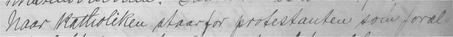Naar katholiken staar for protestanten som foræl-
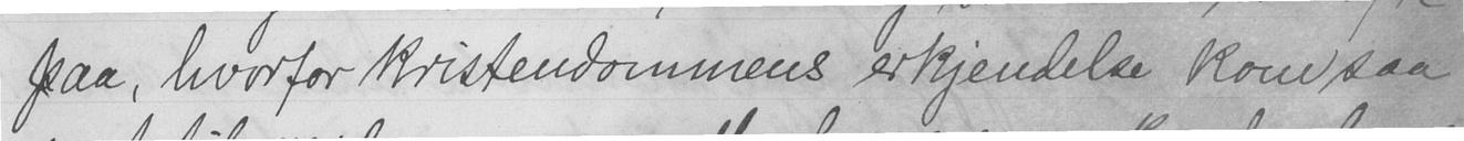paa, hvorfor kristendommens erkjendelse kom saa
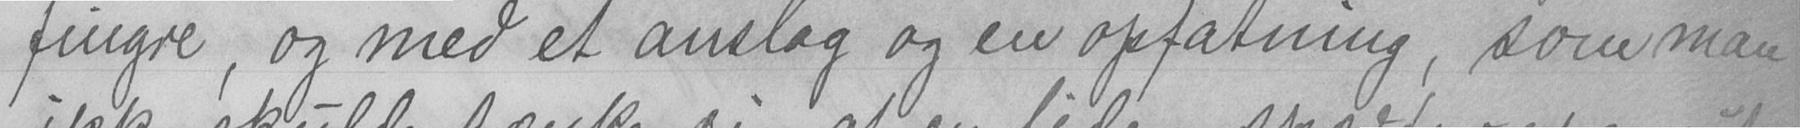fingre, og med et anslag og en opfatning, som man
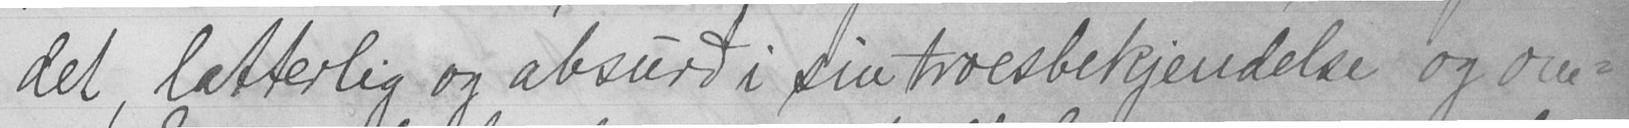det, latterlig og absurd i sin troesbekjendelse og om-
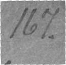167.
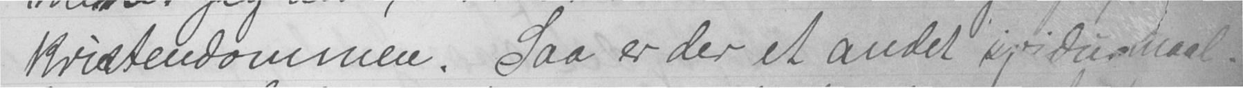kristendommen. Saa er der et andet spørgsmaal
